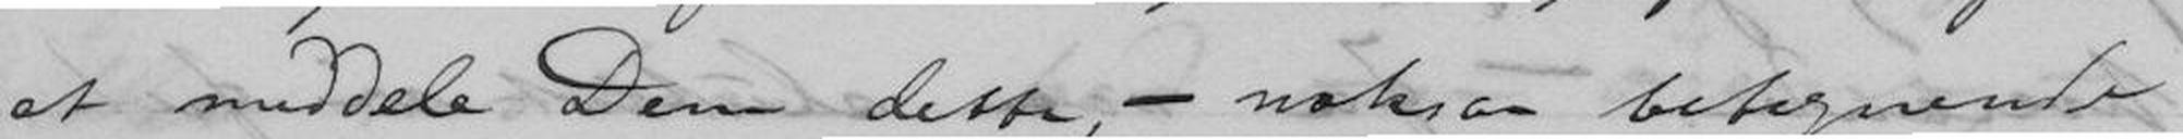at meddele Dem dette, - noksaa betegnende
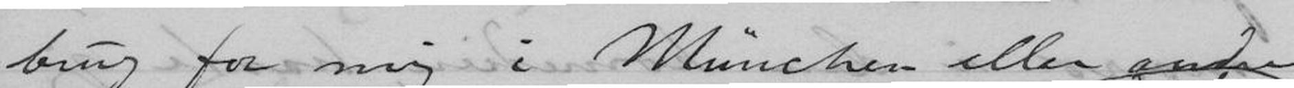brug for mig i München eller andre
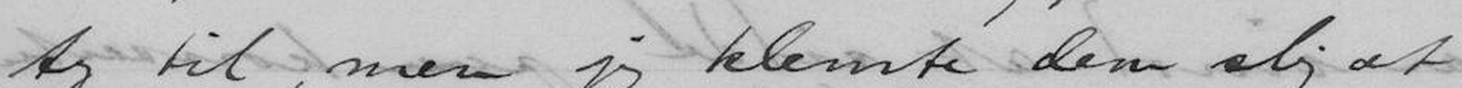ty til, men jeg klemte dem slig at
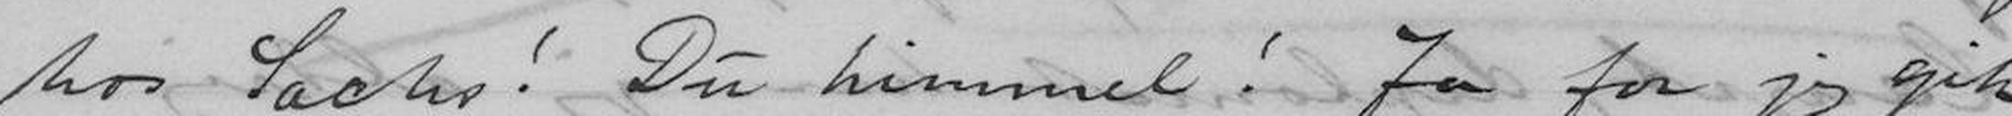hos Sachs! Du himmel! Ja for jeg gik
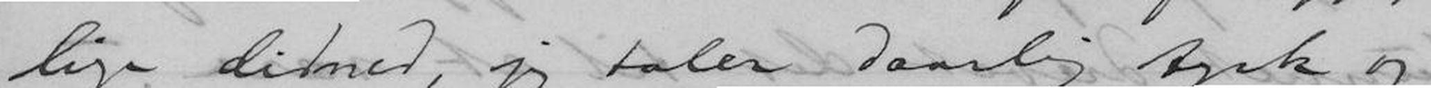lige didned, jeg taler daarlig tysk og
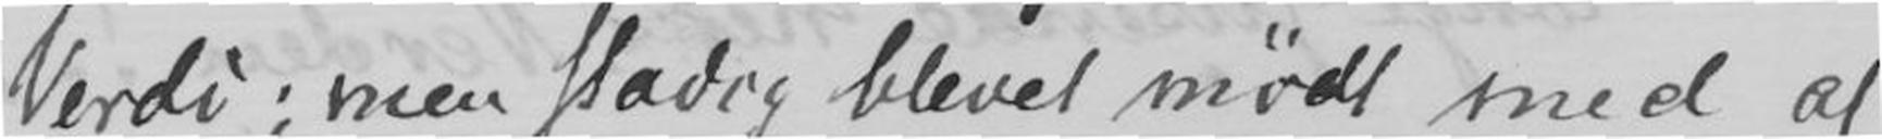Verdi; men stadig blevet mødt med at
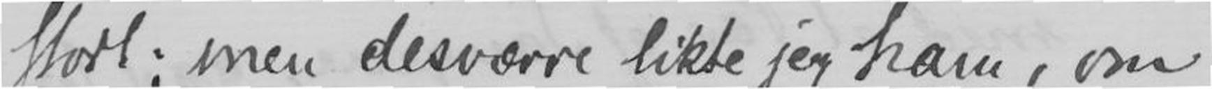stort; men desværre likte jeg ham, om
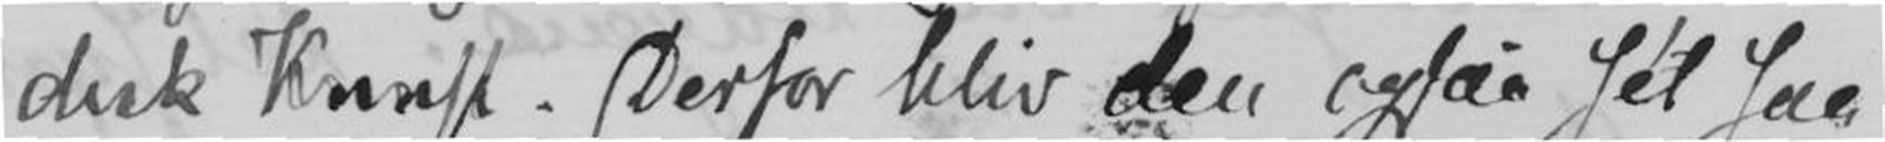disk Kunst. Derfor blir den ogsaa set saa
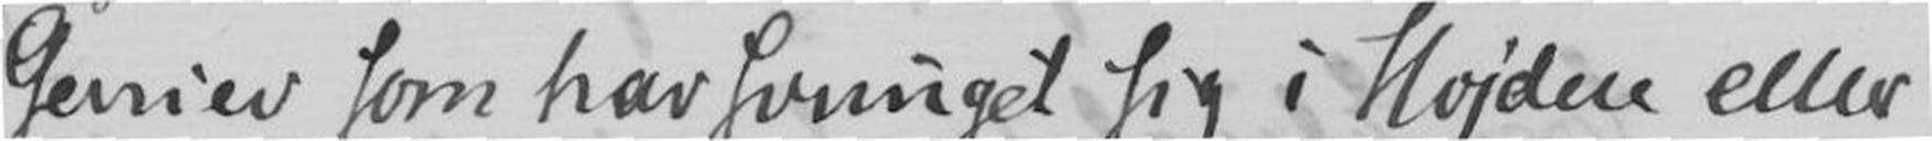Genier som har sunget sig i Højden eller
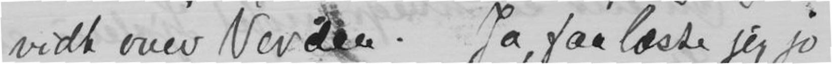vidt over Verden. Ja, saa læste jeg jo
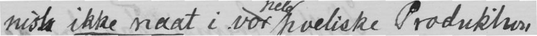nist ikke naat i vor hele poetiske Produktion,
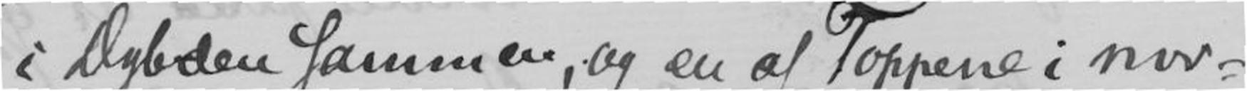i Dybden sammen, og en af Toppene i nor-
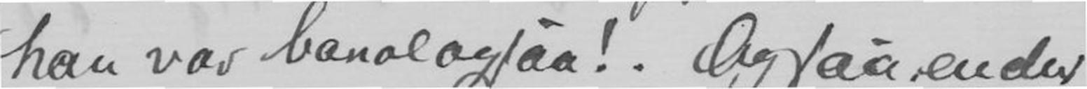han var banal ogsaa!. Og saa ender
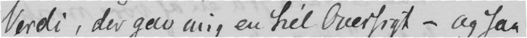Verdi, der gav mig en hel Oversigt - og saa
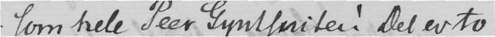- som hele Peer Gyntsuiten! Det er to
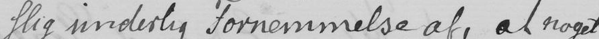slig inderlig Fornemmelse af, at noget
